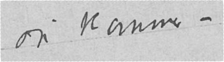du kommer -.
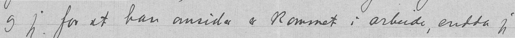og jeg for at han omsider er kommet i arbeide, endda jeg
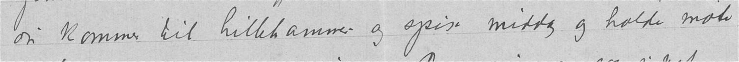du kommer til Lillehammer og spise middag og holde møte
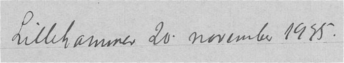Lillehammer 20. november 1935.
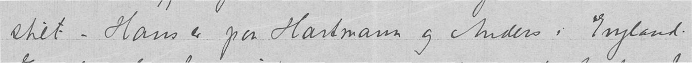stilt – Hans er paa Hartmann og Anders i England.
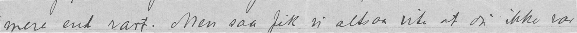mere end rart. Men saa fik vi altsaa vite at du ikke var
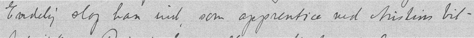Endelig slog han ind, som apprentice ved Austins bil-
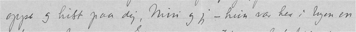oppe og hilst paa dig, Nini og jeg – hun var her i byen en
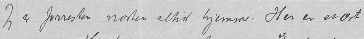Jeg er forresten næsten altid hjemme. Her er svært
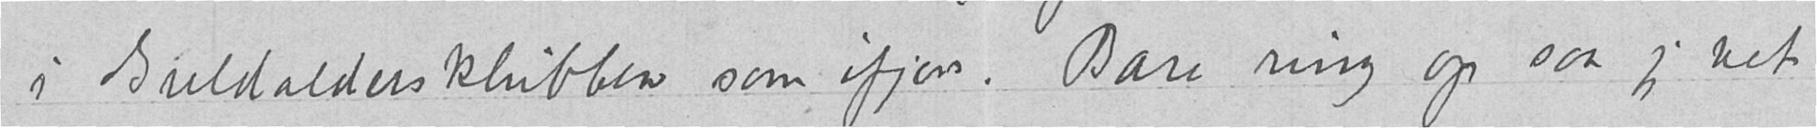i Guldaldersklubben som ifjor. Bare ring op saa jeg vet
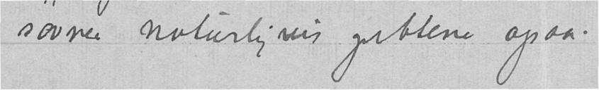savner naturligvis guttene ogsaa.
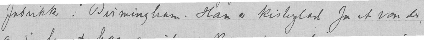fabrikker i Birmingham. Han er kisteglad for at være der,
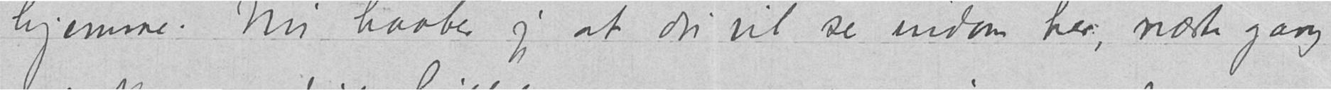hjemme. Nu haaber jeg at du vil se indom her, næste gang
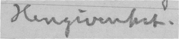Hengivenhet.
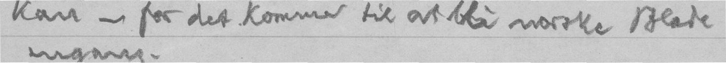kan - for det kommer til at bli norske Blade
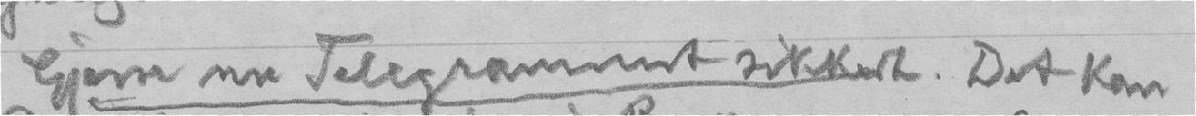Gjem nu Telegrammet sikkert. Det kan
